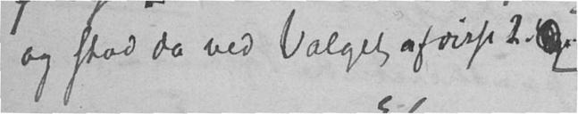og stod da ved Valget af disse 2.]
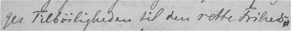ger Tilbøiligheden til den rette Friheds
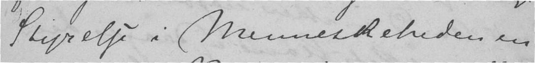Styrelse i Menneskeheden en
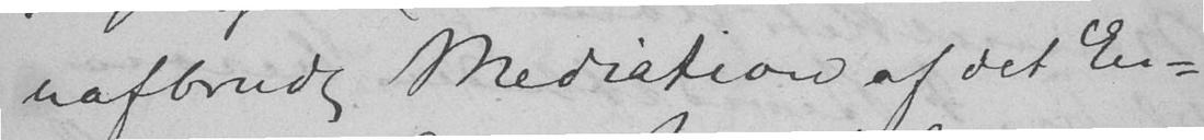uafbrudt Mediation af det En-
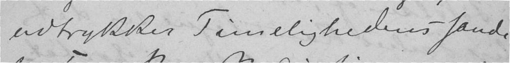udtrykker Timelighedens sande
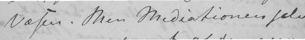Væsen. Men Mediationen selv
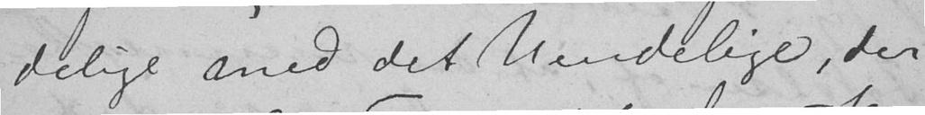delige med det Uendelige, der
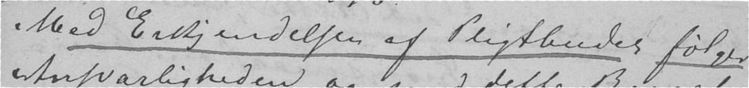Med Erkjendelsen af Pligtbudet følger
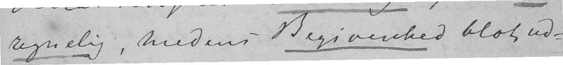regnelig, medens Begivenhed blot ud-
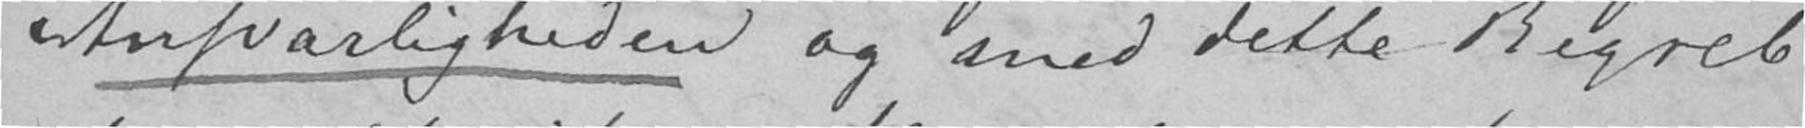Ansvarligheden og med dette Begreb
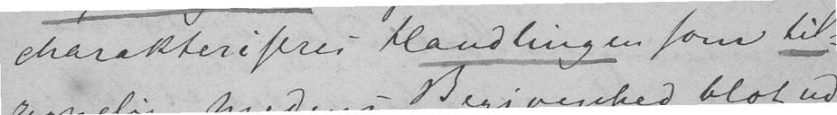charakteriseres Handlingen som til-
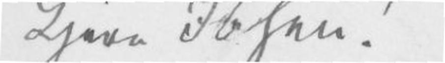Kjere Ibsen!
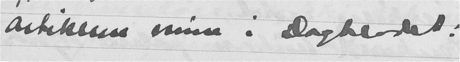artiklene mine i Dagbladet!
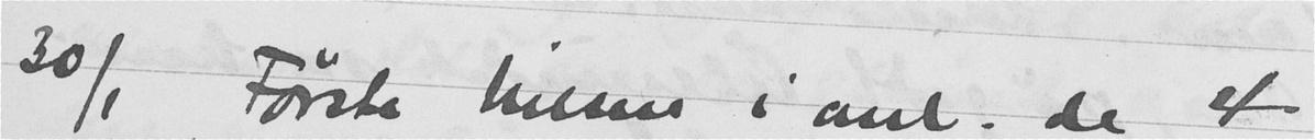30/1 Første hilsen i anl. de 4
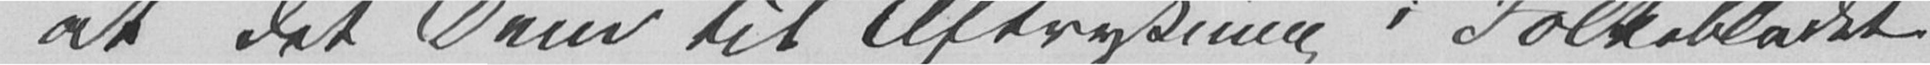at det Dem til Aftrykning i Folkebladet
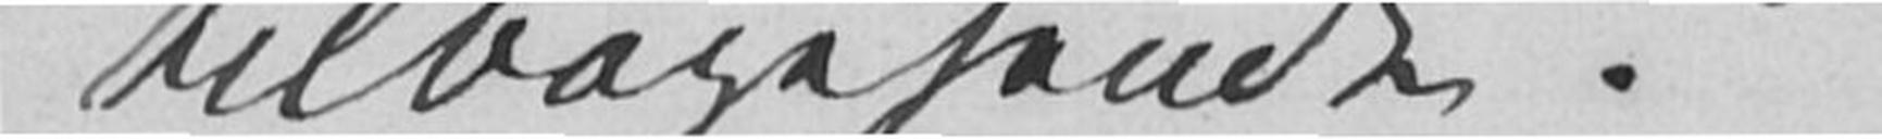tilbagesendt.
